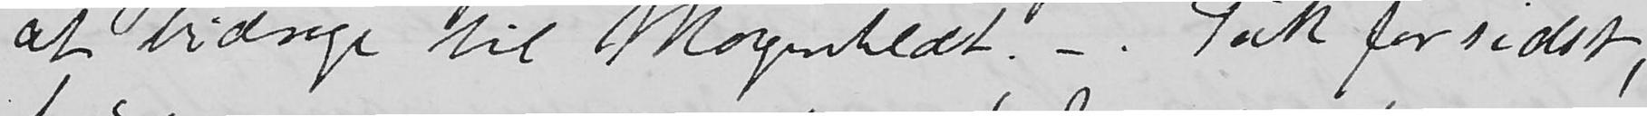at bidrage til Morgenbladt. - Tak for sidst,
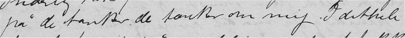fa de tanker de tænker om mig. I det hele
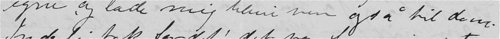egne og lade mig blive ven også til dem.
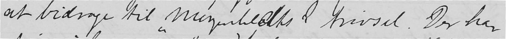at bidrage til "Morgenbladets" trivsel. Der har
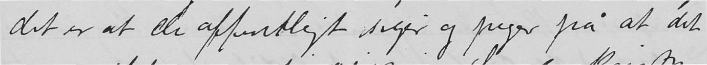det er at de offentligt siger og peger på at det
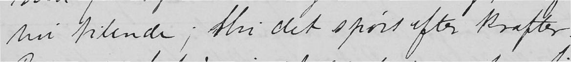vei tilende; thi det spørs efter kræfter.
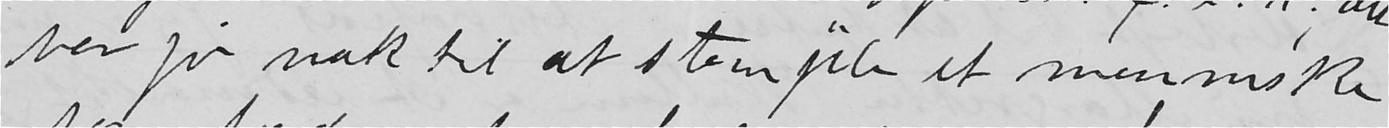er jo nok til at stemple et menneske
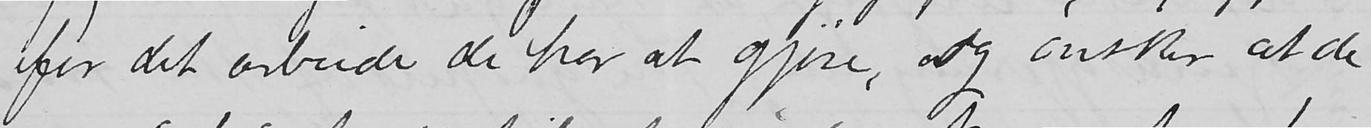for det arbeide de har at gjøre, og ønsker, at de
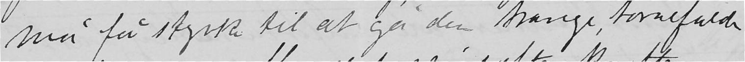må få styrke til at gå den trange, tornefulde
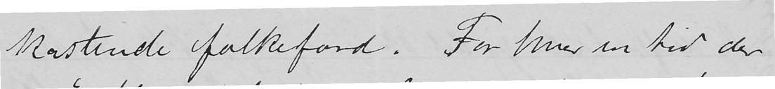kastende folkefærd. For hver en tid der
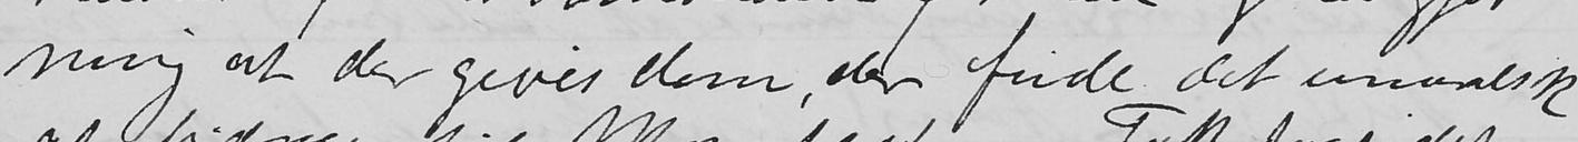ning at der gives dem, der finde det umoralsk
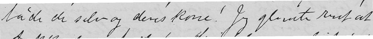både de selv og deres kone! Jeg glemte rent at
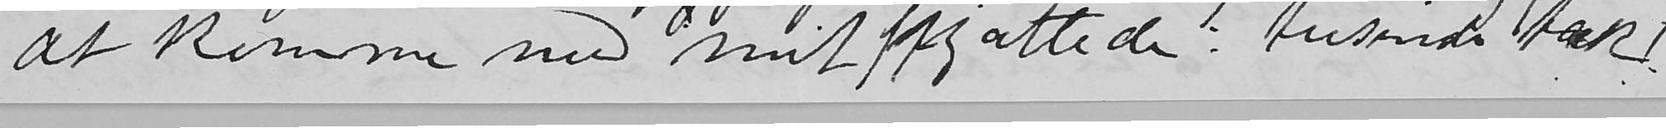at komme med mit pjattede: tusinde tak!
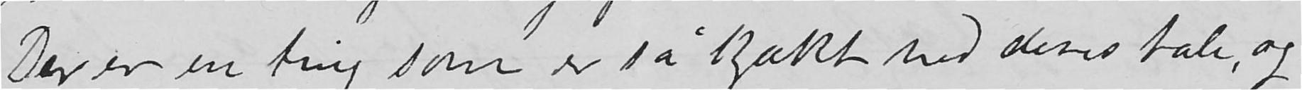Der er en ting som er så kjækt med deres tale, og
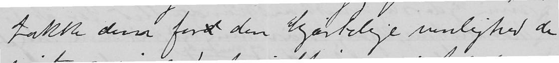takke dem ford den hjærtelige venlighed de
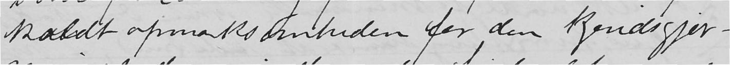kaldt opmærksomheden for den kjendsgjer-
